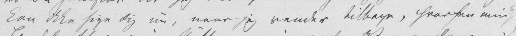kan ikke sige Dig nu, naar jeg vender tilbage, hvorhen min
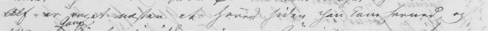Alf er voxet næsten et Hoved siden han kom herned, og
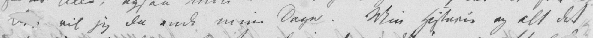der vil jeg da ende mine Dage. Min Historie og alt det
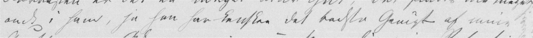ondt i ham, ja han har kanskee det bedste Gemyt af mine
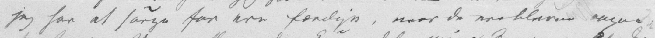jeg har at sørge for ere færdige, naar de ere blevne voxne,
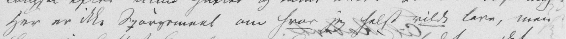Her er ikke Spørgsmaal om hvor jeg helst vilde leve, men
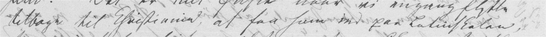tilbage til Christiania, at faa ham ind paa Latinskolen,
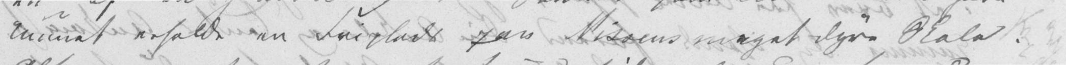kunnet erholde en Friplads paa Nissens meget dyre Skole.
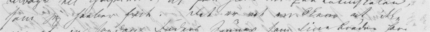som jeg haaber frit. Det er ret en Skam at ikke
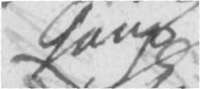Gang
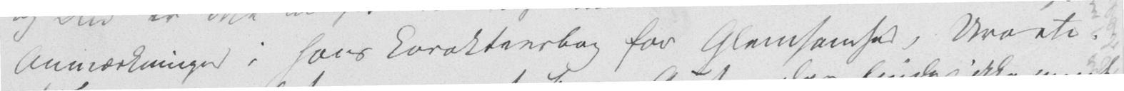Anmærkninger i hans Carakteerbog for Glemsomhed, Uro etc.
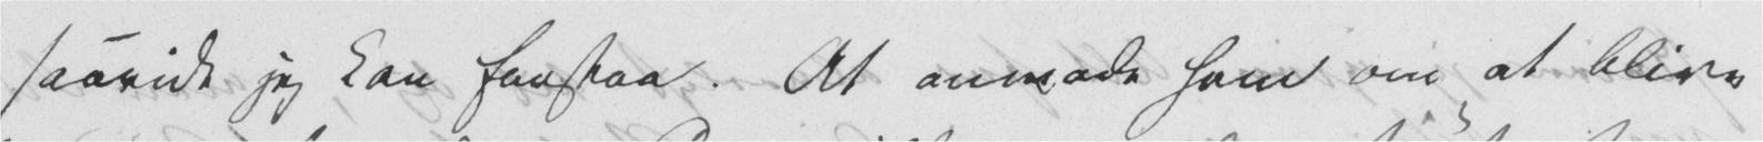saavidt jeg kan forstaa. At anmode ham om at blive
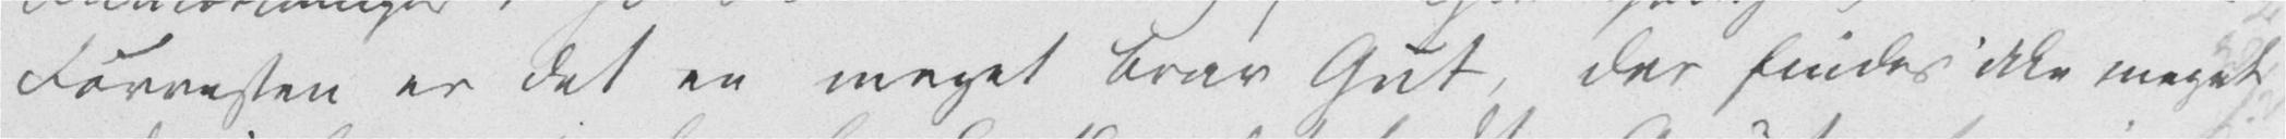Forresten er det en meget brav Gut, der findes ikke meget
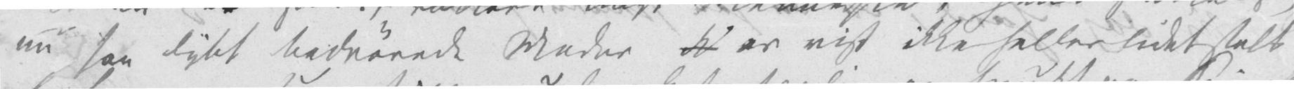nu saa dybt bedrøvede Moder er vist ikke heller lidet stolt
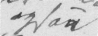ogsaa
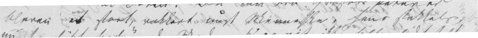bleven et stort, vakkert ungt Menneske, hans stakkels,
